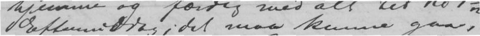Eftermiddag; det maa kunne gaa,
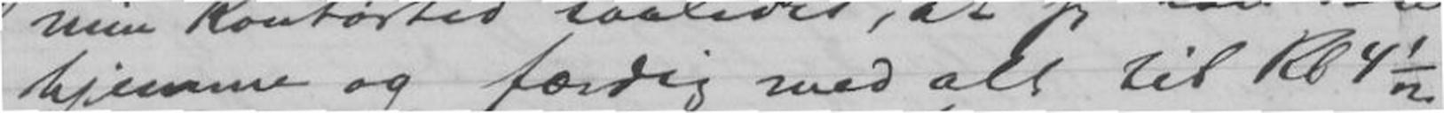hjemme og færdig med alt til Kl 4 ½
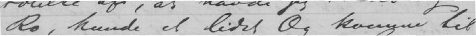Ro, kunde et lidet Og komme til
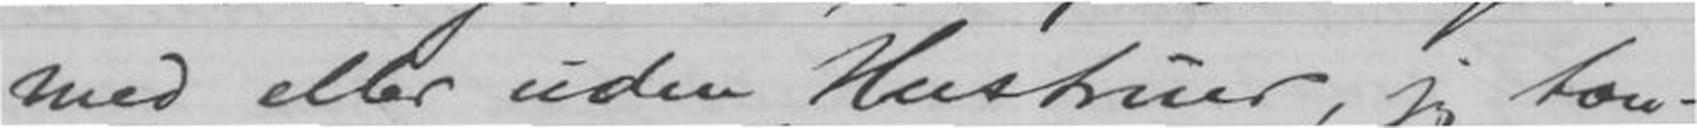med eller uden Hustruer, jeg tæn-
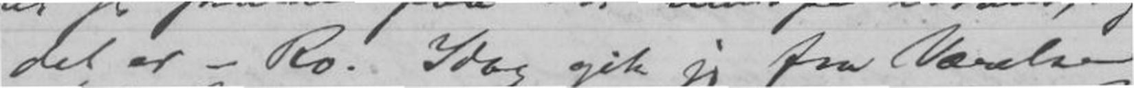det er - Ro. Idag gik jeg fra Værelse
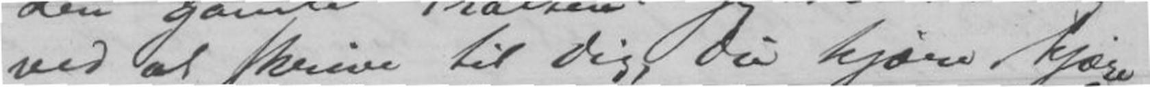ved at skrive til Dig, Du kjære kjære
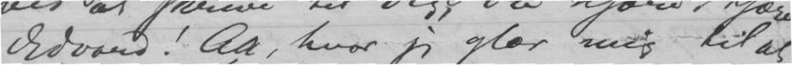Edvard! Aa, hvor jeg glær mig til at
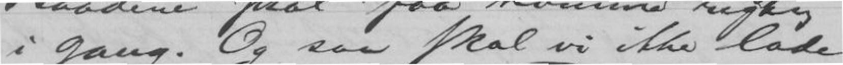i gang. Og saa skal vi ikke lade
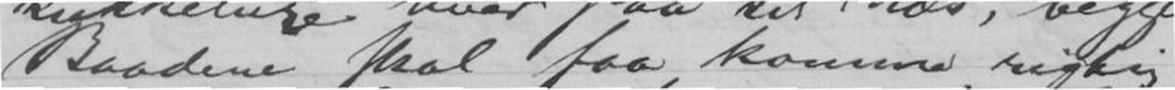Baadene skal faa komme rigtig
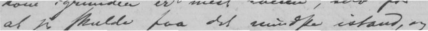at jeg skulde faa det mindste istand, og
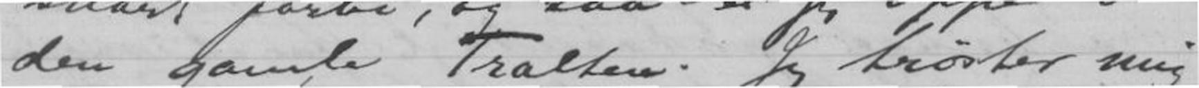den gamle Tralten. Jeg trøster mig
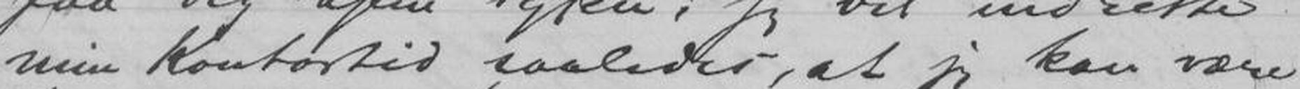min Kontortid saaledes, at jeg kan være
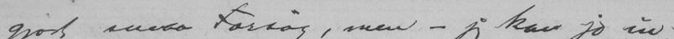gjort smaa Forsøg, men - jeg kan jo in-
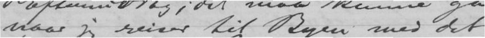naar jeg reiser til Byen med det
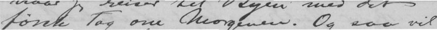første Tog om Morgenen. Og saa vil
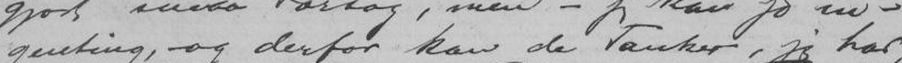genting, og derfor kan de Tanker, jeg har,
